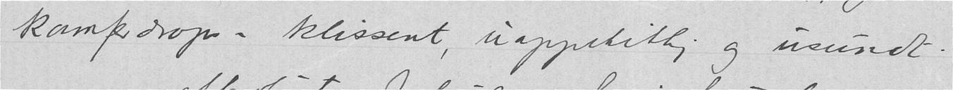kamferdrops – klissent, uappetitlig og usundt.
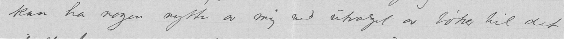kan ha nogen nytte av mig ved utvalget av bøker til det
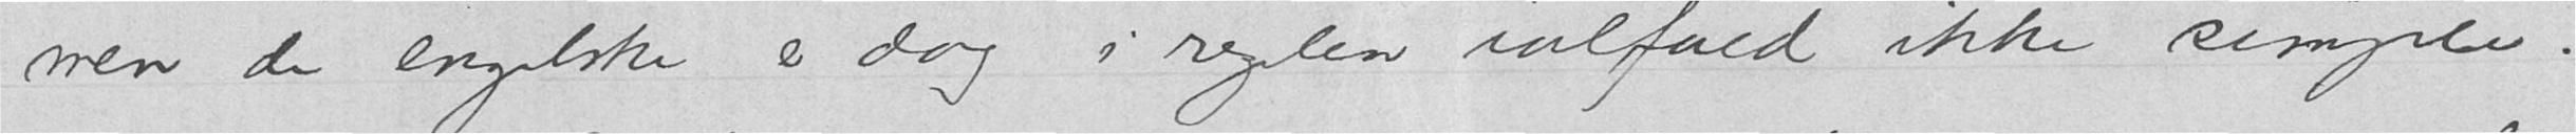men de engelske er dag i regelen ialfald ikke simple.
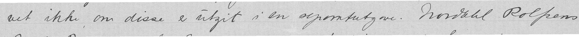vet ikke om disse er utgit i en separatutgave. Nordahl Rolfsens
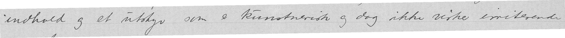indhold og et utstyr som er kunstnerisk og dog ikke virker irriterende
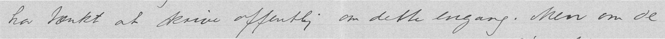har tænkt at skrive offentlig om dette engang. Men om De
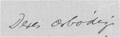Deres ærbødige
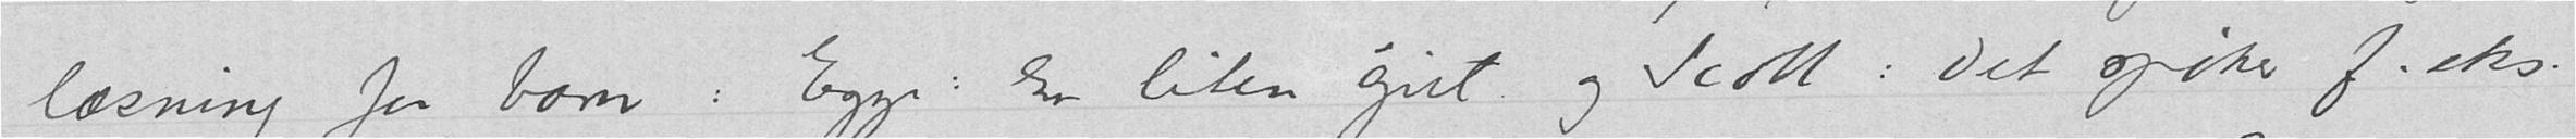læsning for barn: Egge: En liten gut og Scott: Det spøker f.eks.
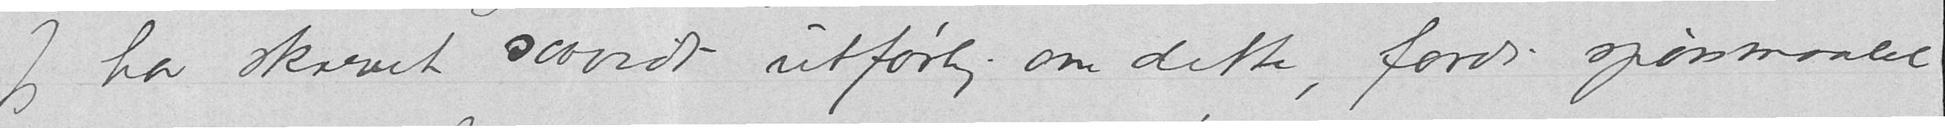Jeg har skrevet saavidt utførlig om dette, fordi spørsmaalet
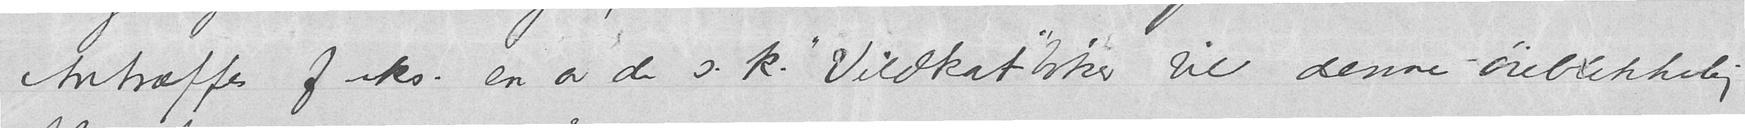Antræffes f eks. en av de s.k. "Vildkat"bøker vil denne øieblikkelig
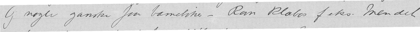Og nogle ganske faa barnebøker – Ravn Klæbos f eks. Men det
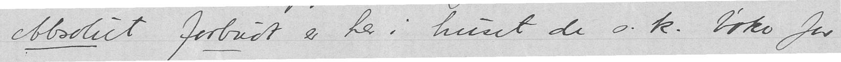Absolut forbudt er her i huset de o.k. bøke for
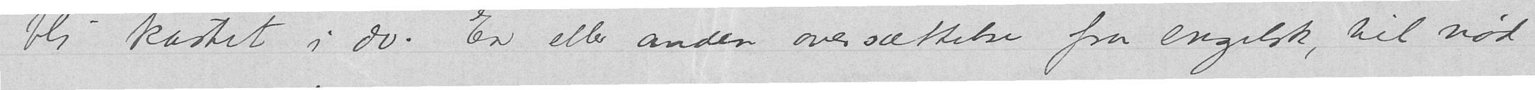bli kastet i do. En eller anden oversættelse fra engelsk, til nød
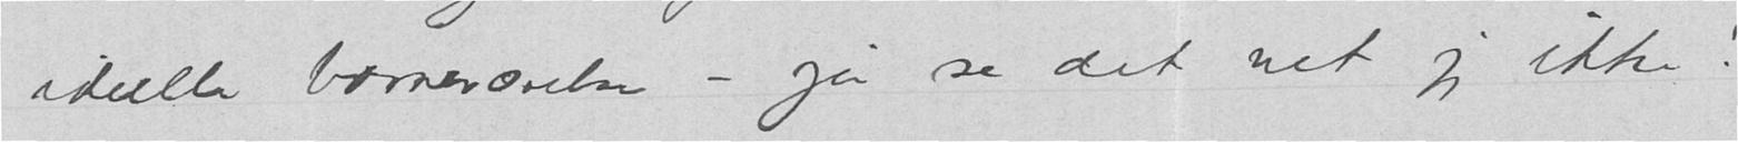idelle børneværelse – ja se det vet jeg ikke!
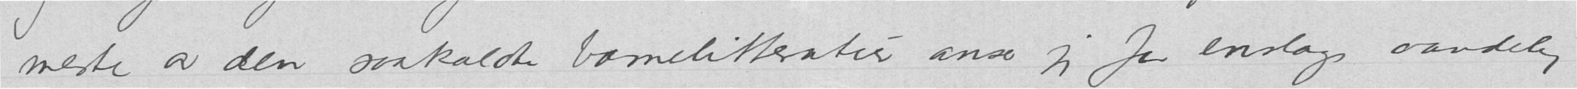meste av den saakaldte barnelitteratur anser jeg for enslags aandelig
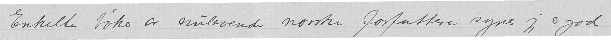Enkelte bøker av nulevende norske forfattere synes jeg er god
